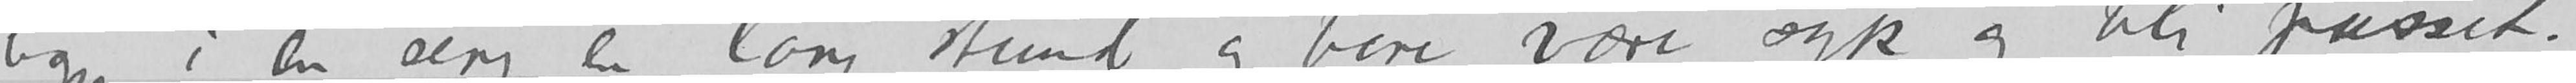ligge i en seng en lang stund og bare være syk og bli passet.
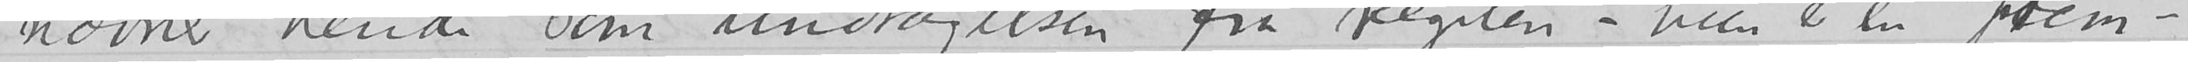nævner hende som undtagelsen fra regelen – hun er en frem-
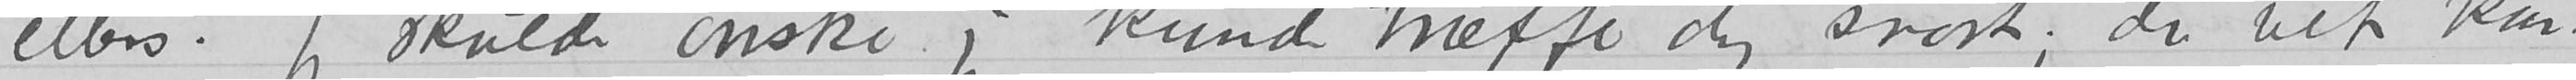ellers. Jeg skulde ønske jeg kunde træffe dig snart; du vet kan-
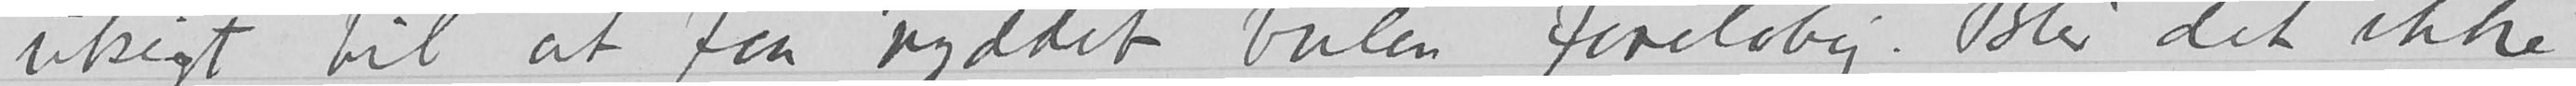utsigt til at faa ryddet bulen foreløbig. Blir det ikke
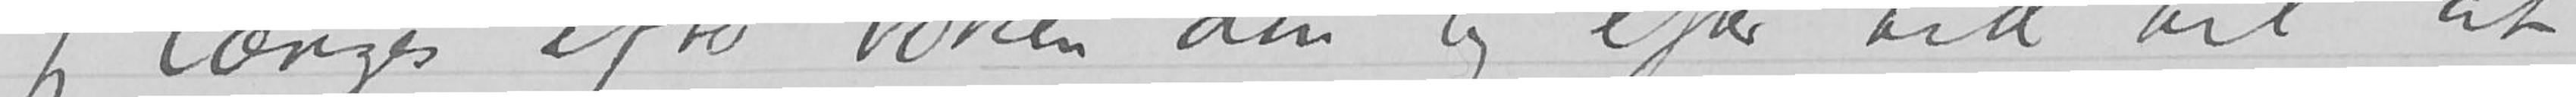Jeg længes efter boken din og efter tid til at
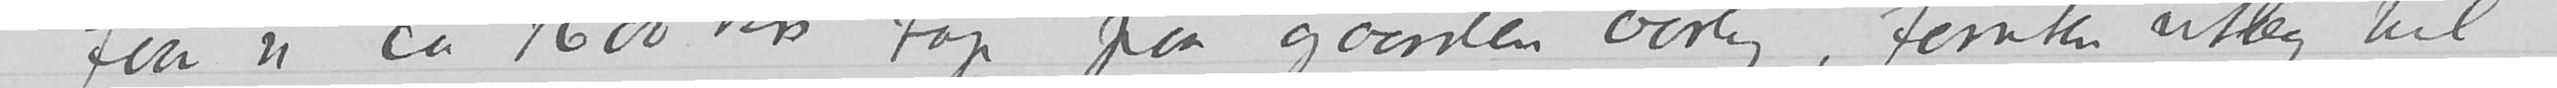faar vi ca 1600 krs tap paa gaarden aarlig, foruten utlæg til
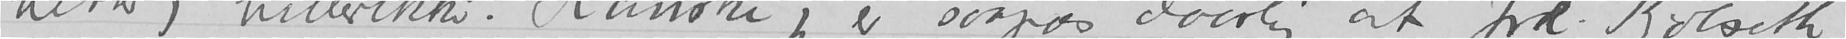hetter) hellerikke. Kanske jeg er saapas daarlig at frk. Kjølseth
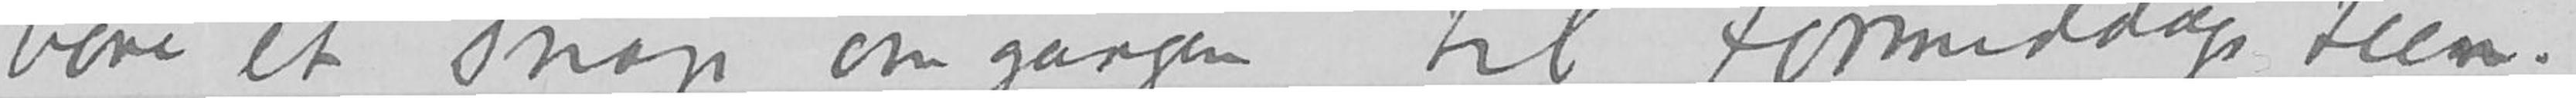bare et snap om gangen til formiddags teen.
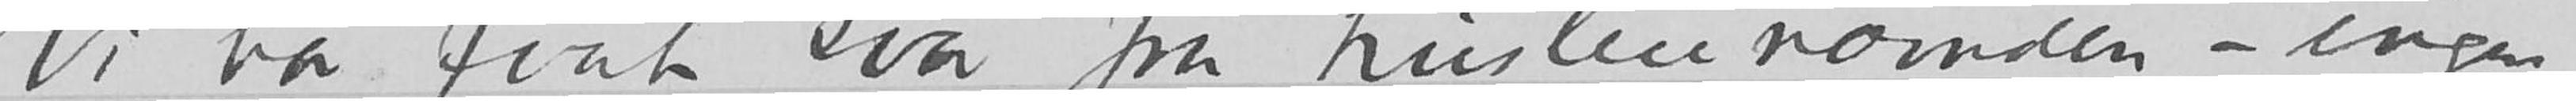Vi har faat svar fra husleienæmden – ingen
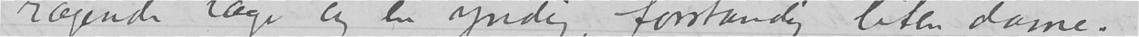ragende læge og en yndig, forstandig liten dame.
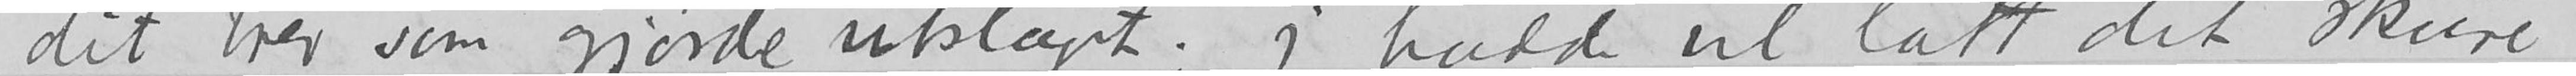dit brev som gjorde utslaget; jeg hadde vel latt det skure
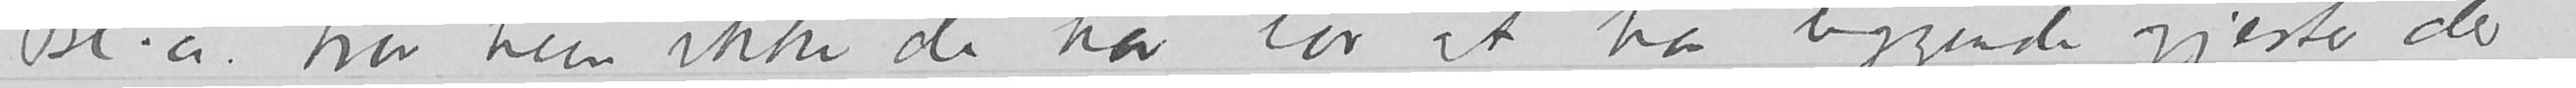Bl.a. tror hun ikke de har lov at ha liggende gjester der
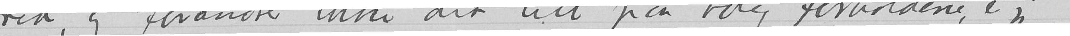fred, og forandrer ikke det litt paa bolig forholdene, er jeg
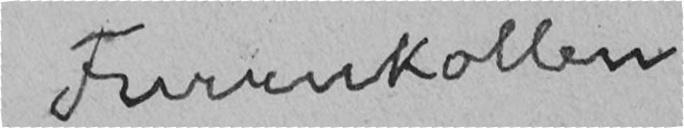Furukollen
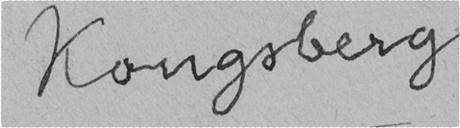Kongsberg
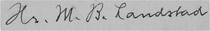Hr. M. B. Landstad
